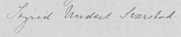Sigrid Undset Svarstad.
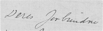Deres forbundne
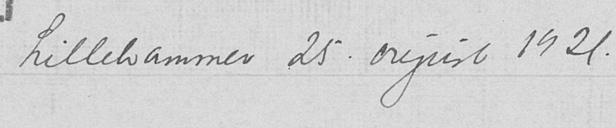Lillehammer 25. august 1921.
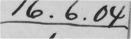16.6.04
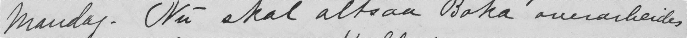Mandag. Nu skal altsaa Boka overarbeides
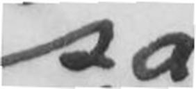så
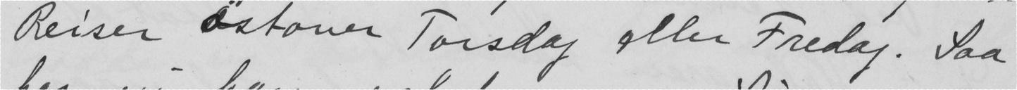Reiser Østover Torsdag eller Fredag. Saa
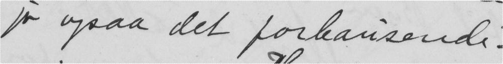jo ogsaa det forbausende
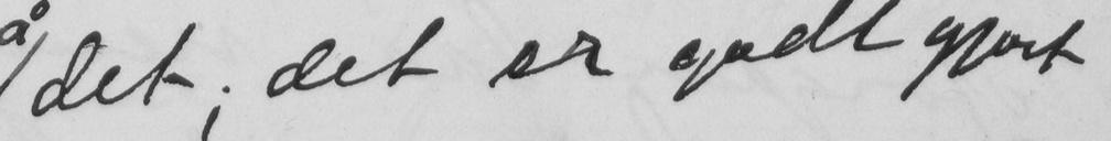det, det er godt gjort
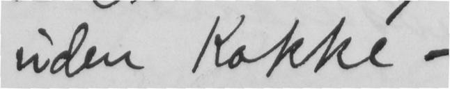uden Kokke -
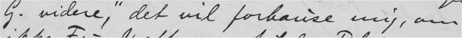G. videre, "det vil forbause mig, om
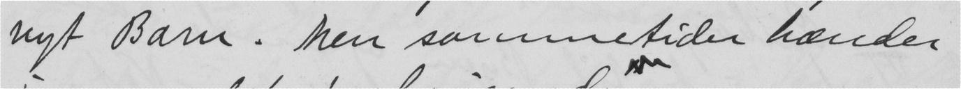nyt Barn. Men sommetider hænder
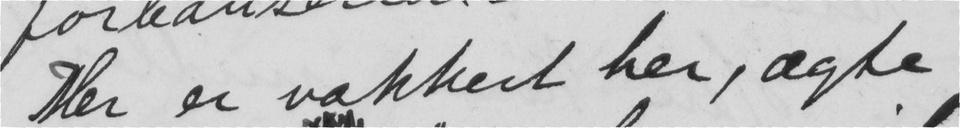Her er vakkert her, ægte
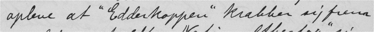opleve at "Edderkoppen" Krabber sig frem
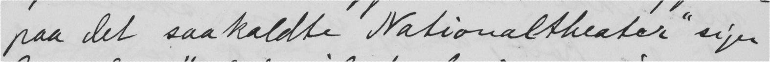paa det saakaldte Nationaltheater" siger
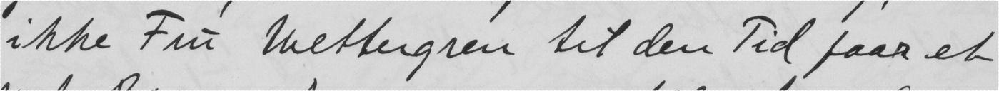ikke Fru Wettergren til den Tid faar et
